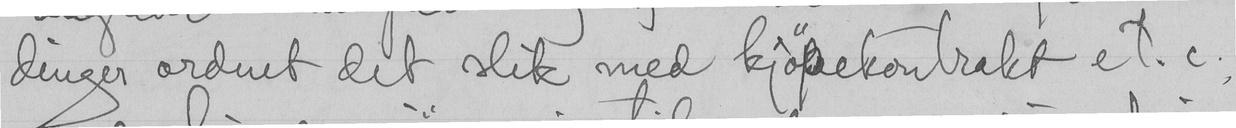dinger ordnet det slik med kjøpekontrakt et.c.
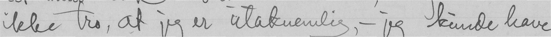ikke tro, at jeg er utaknemlig, - jeg kunde have
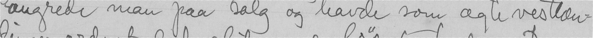angrede man paa salg og havde som ægte vestlæn-
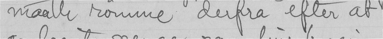maatte rømme derfra efter at
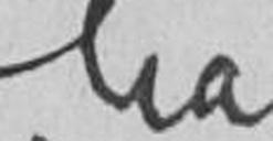ha
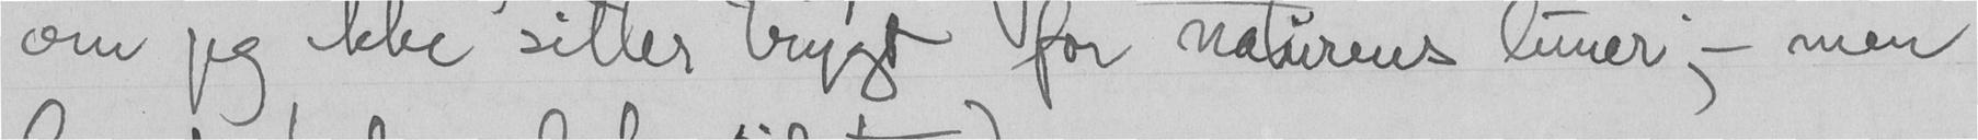om jeg ikke sitter trygt for naturens luner, - men
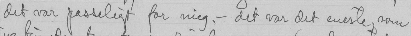det var passeligt for mig, - det var det eneste som
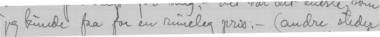jeg kunde faa for en rimelig pris; - (andre steder
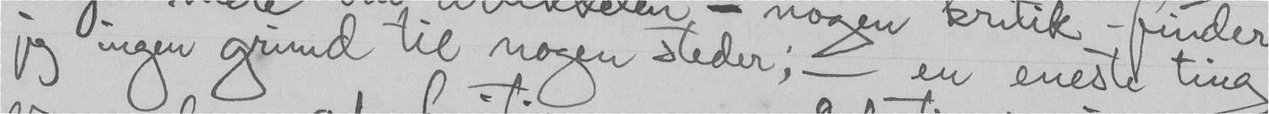jeg ingen grund til nogen steder; - en eneste ting
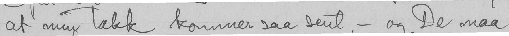at min takk kommer saa sent - og De maa
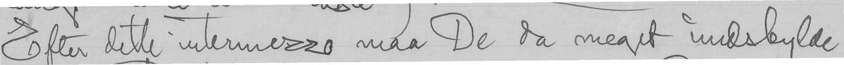Efter dette intermezzo maa De da meget undskylde
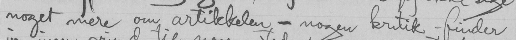noget mere om artikkelen - nogen kritik - pender
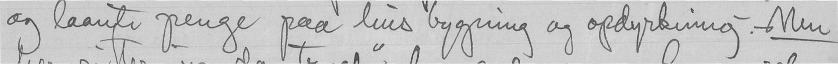og laante penge paa hus bygning og opdyrkning. - Men
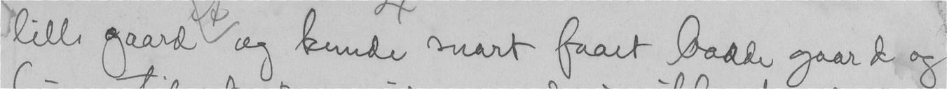lille gaard - og kunde snart faaet baade gaard og
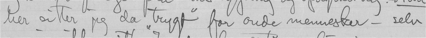har sitter jeg da "trygt" for onde mennesker - selv
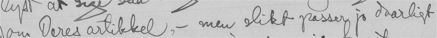om Deres arlikkel, - men slikt passer jo daarligt
